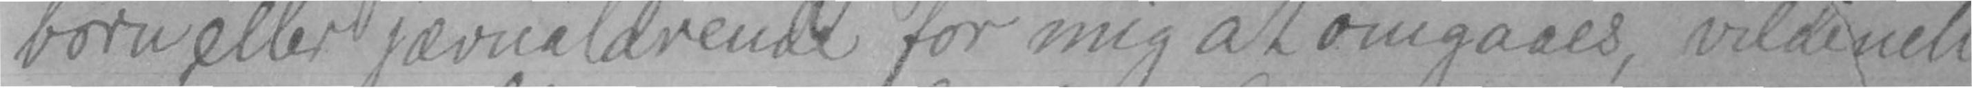børn eller jævnaldrende for mig at omgaaes, vilde
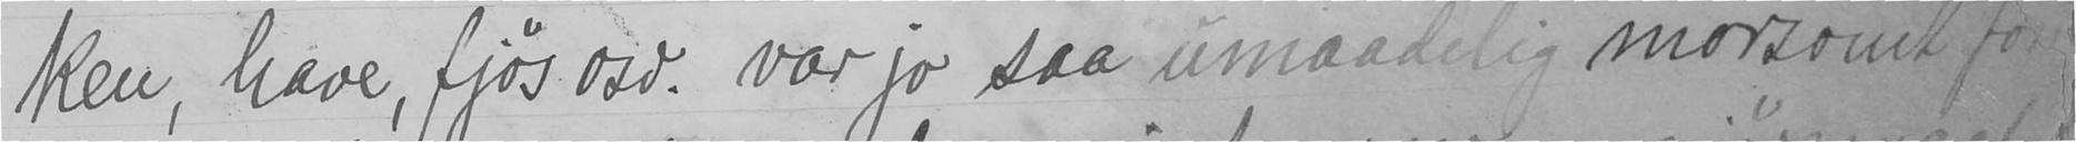ken, have, fjøs osv. var jo saa umaadelig morsomt for
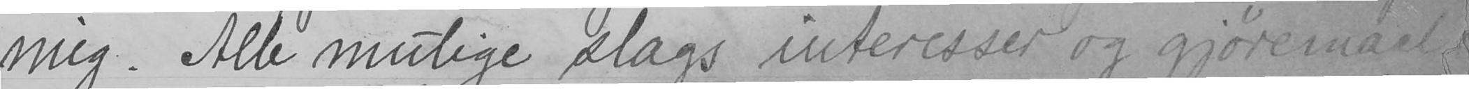mig. Alle mulige slags interesser og gjøremaal
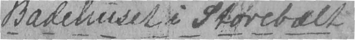Badehuset i Størebælt
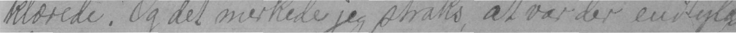klærede. Og det merkede jeg straks, at var der end
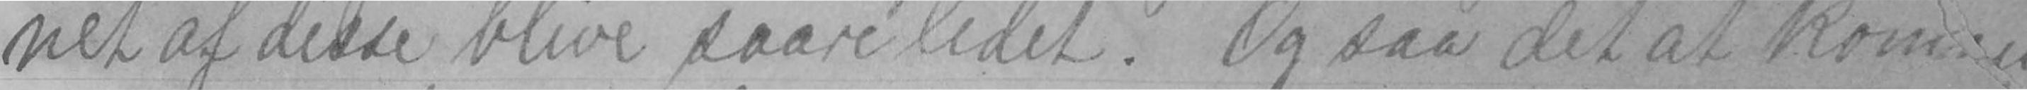net af disse blive saare lidet. Og saa det at kom
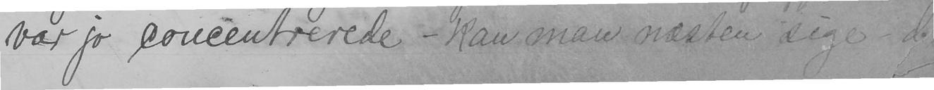var jo concentrerede - kan man næsten sige - d.
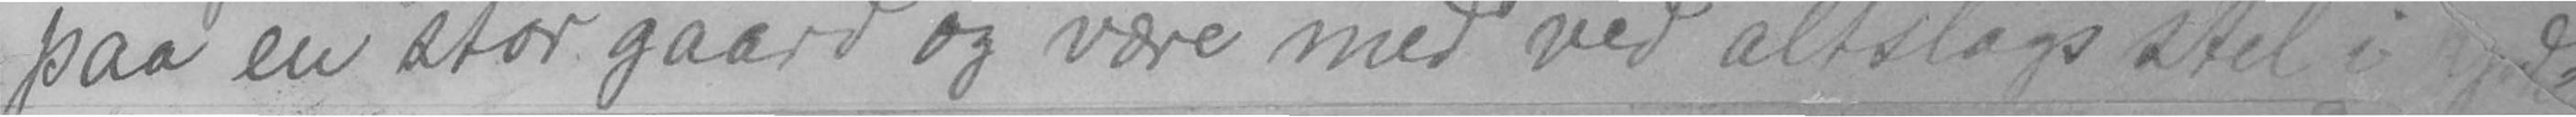paa en stor gaard og være med ved altslags stel i
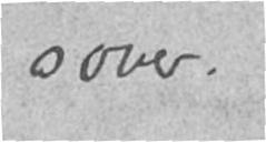sover.
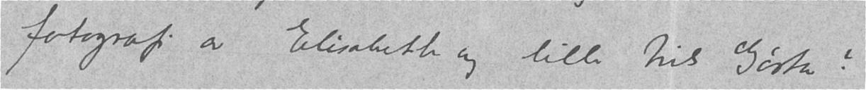fotografi av Elisabeth og lille Nils Gøsta?
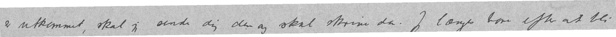er utkommet, skal jeg sende dig den og skal skrive da. Jeg længes bare efter at bli
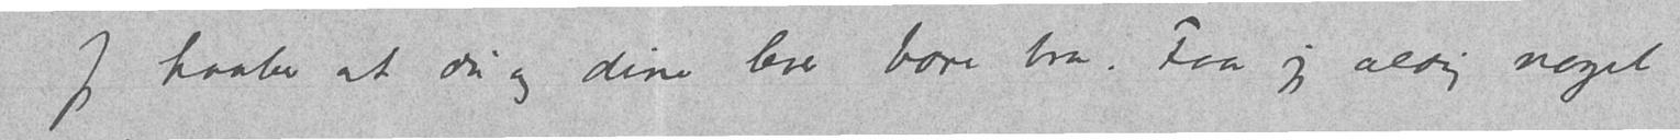Jeg haaber at du og dine lever bare bra. Faar jeg aldrig noget
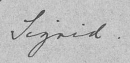Sigrid.
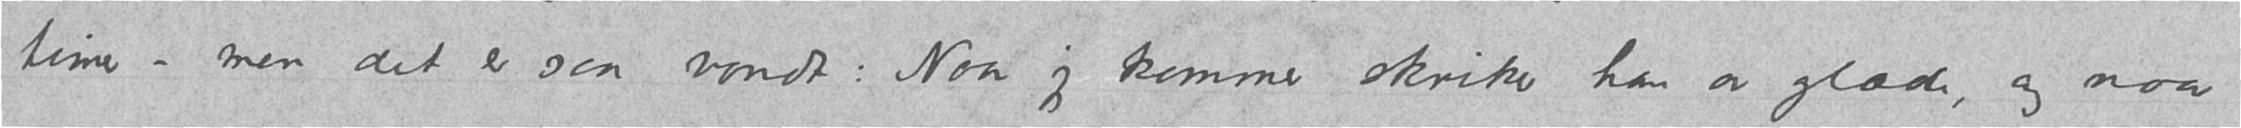timer - men det er saa vondt: Naar jeg kommer skriker han av glæde, og naar
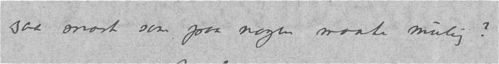saa snart som paa nogen maate mulig?
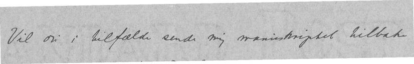Vil du i tilfælde sende mig manuskriptet tilbake
